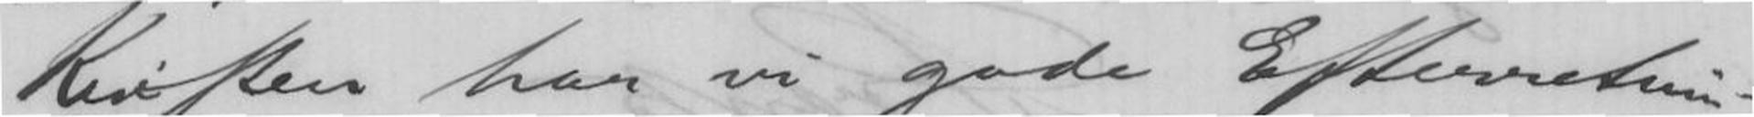Kristen har vi gode Efterretnin-
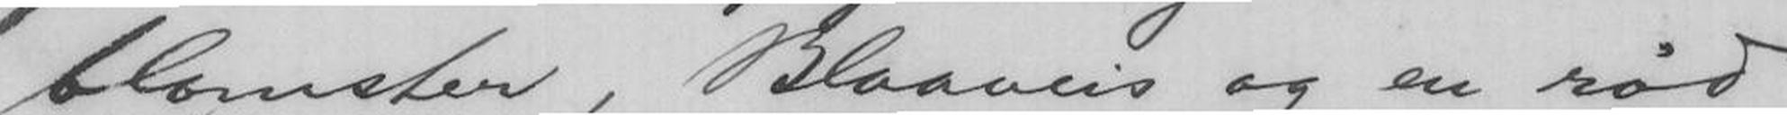blomster, Blaaveis og en rød
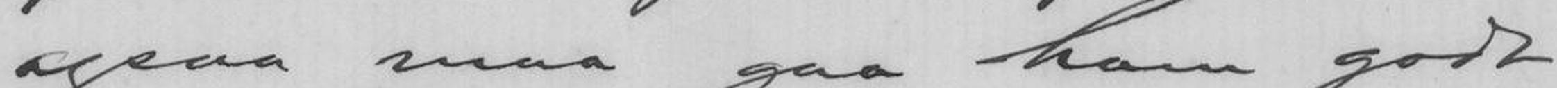ogsaa maa gaa ham godt
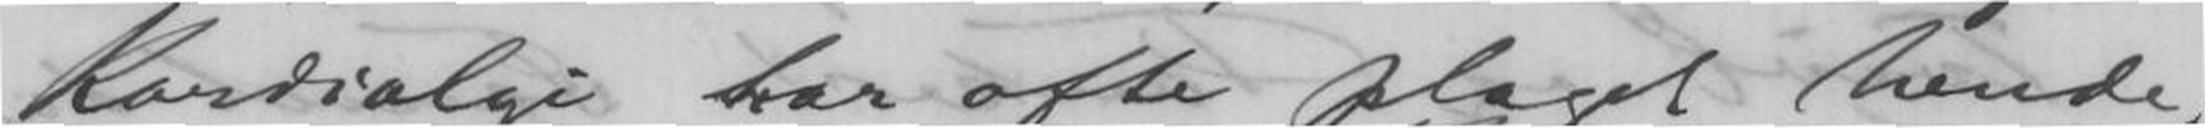Kardialgi har ofte plaget hende,
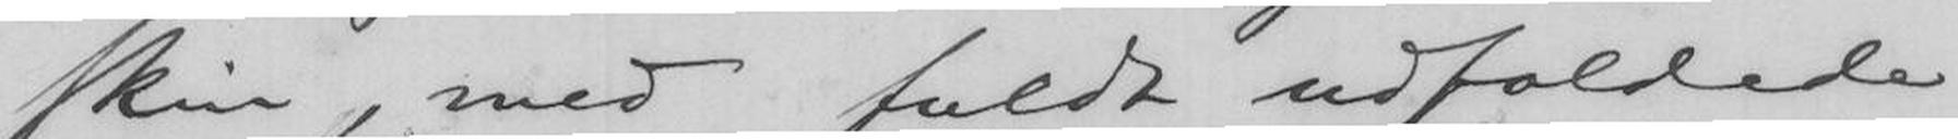skin, med fuldt udfoldede
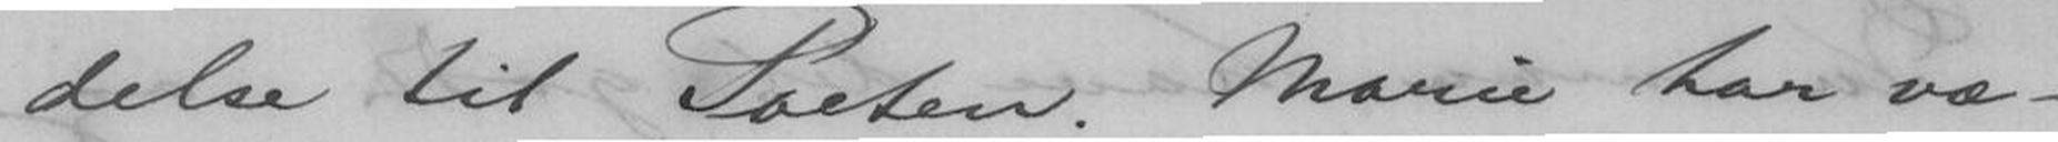delse til Poeten. Marie har væ-
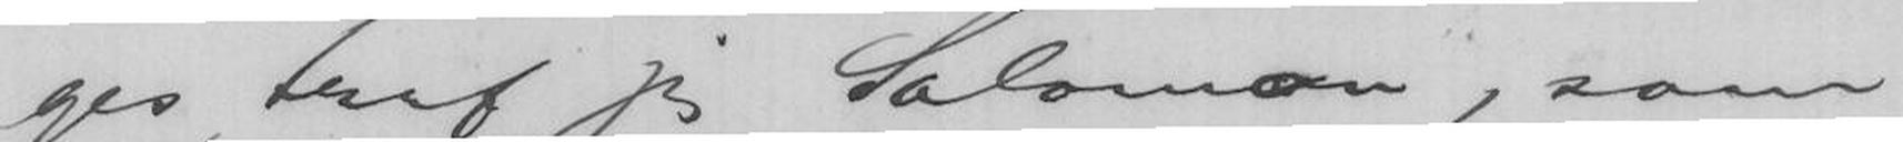ges traf jeg Salomon, som
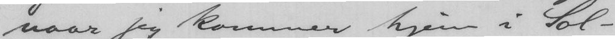naar jeg kommer hjem i Sol-
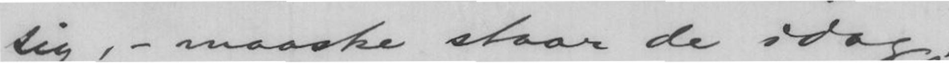sig, - maaske staar de idag,
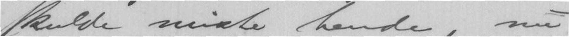skulde miste hende, nu
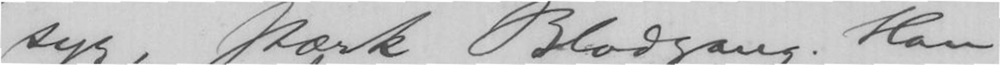syg, stærk Blodgang. Han
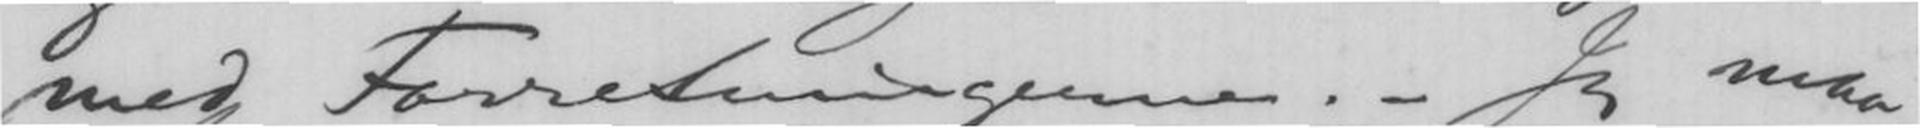med Forretningerne. - Jeg maa
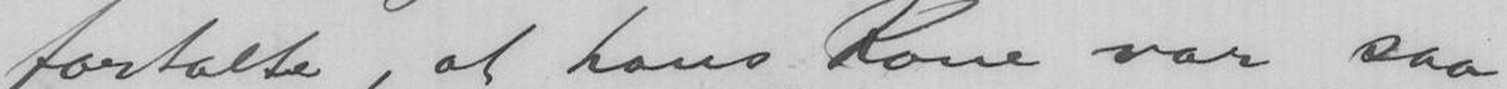fortalte, at hans Kone var saa
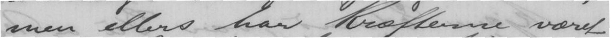men ellers har Kræfterne været
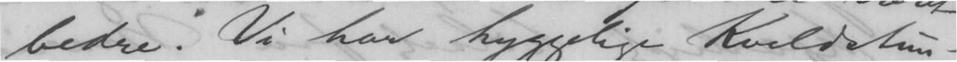bedre. Vi har hyggelige Kveldstun-
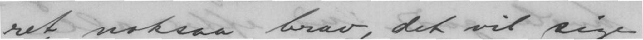ret noksaa brav, det vil sige
